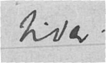tider.
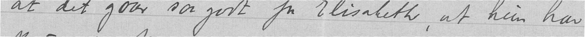at det gaar saa godt for Elisabeth, at hun har
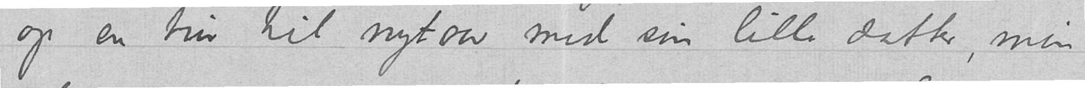op en tur til nytaar med sin lille datter, min
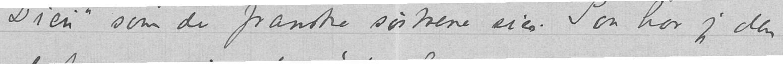Dieu" som de franske søstrene sier. Saa har jeg den
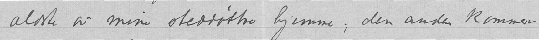ældste av mine steddøttre hjemme, den anden kommer
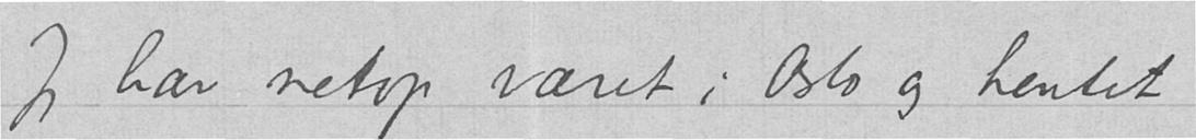Jeg har netop været i Oslo og hentet
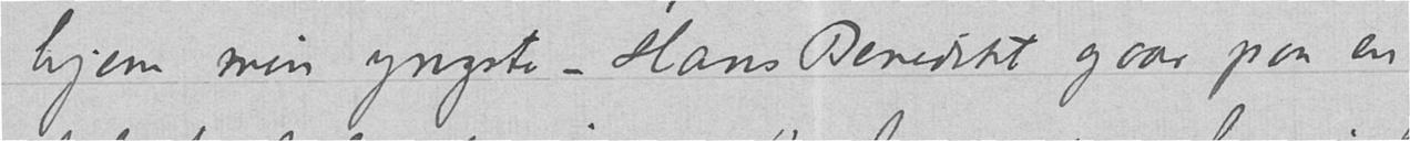hjem min yngste - Hans Benedikt gaar paa en
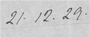21.12.29.
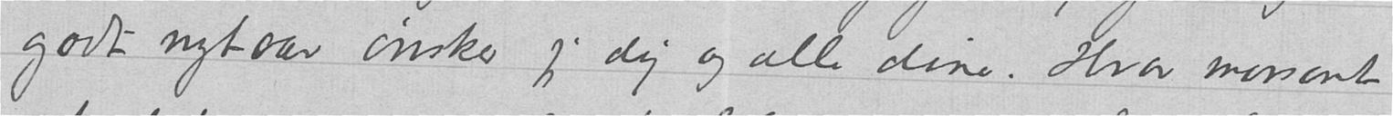godt nytaar ønsker jeg dig og alle dine. Hvor morsomt
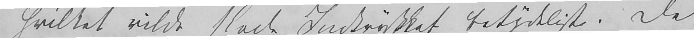hvilket vilde skade Indtrykket betydeligt. De
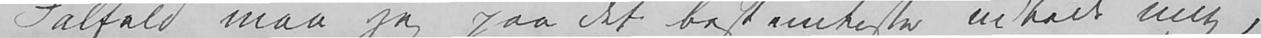Ialfald maa jeg paa det bestemteste udbede mig,
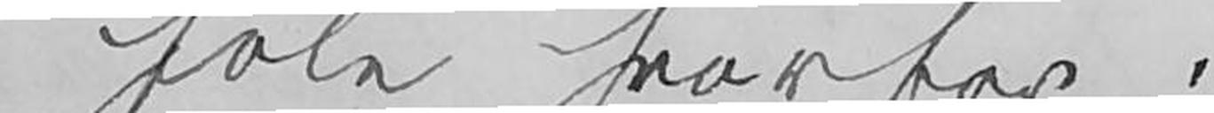føle hvorfor.
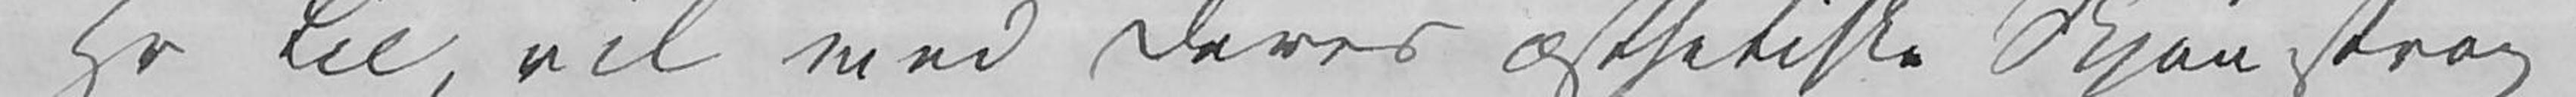Hr Lie, vil med Deres æsthetiske Skjøn strax
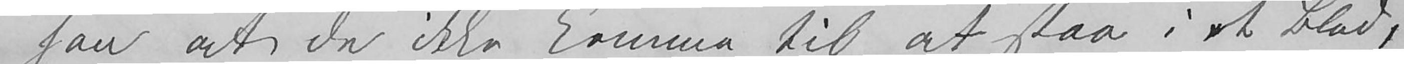saa at de ikke komme til at staa i et Blad,
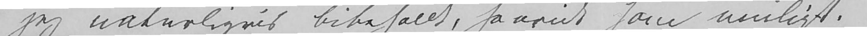jeg naturligvis bibeholdt, saavidt som muligt.
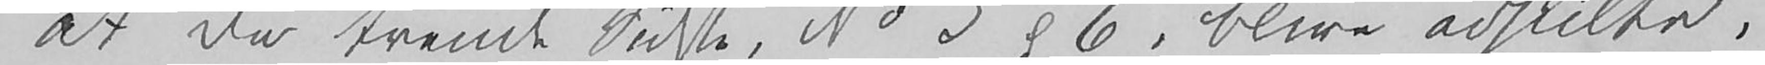at de tvende Sidste, No 5 & 6, blive adskilte,
